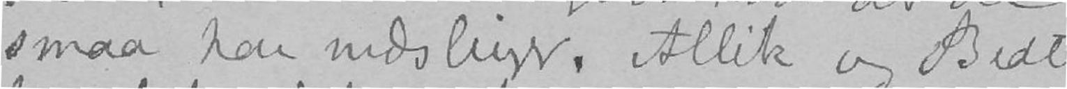smaa har mæslinger. Allik og Beate
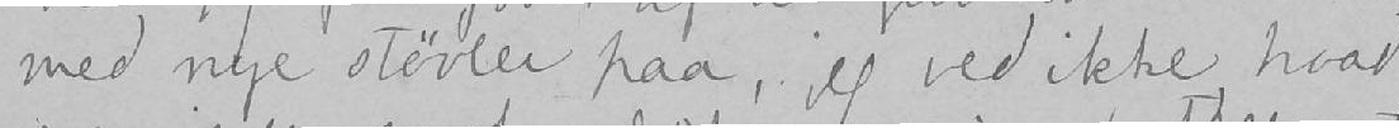med nye støvler paa, jeg ved ikke hvad
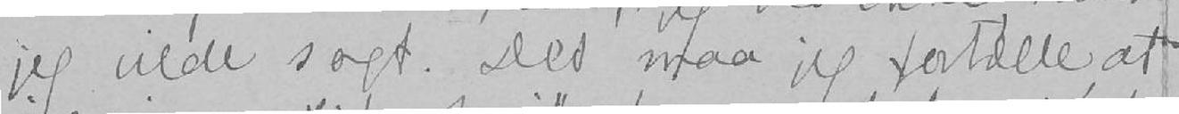jeg vilde sagt. Det maa jeg fortælle, at
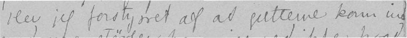blev jeg forstyrret af at gutterne kom ind
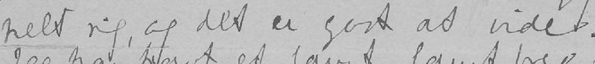helt rig, og det er godt at vide.
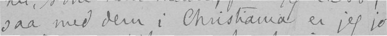saa med dem i Christiania er jeg jo
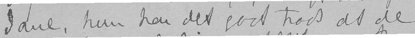Jane, hun har det godt trods at de
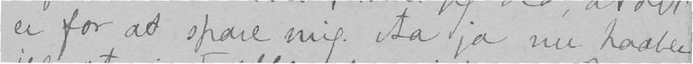er for at spare mig. Aa ja nu haaber
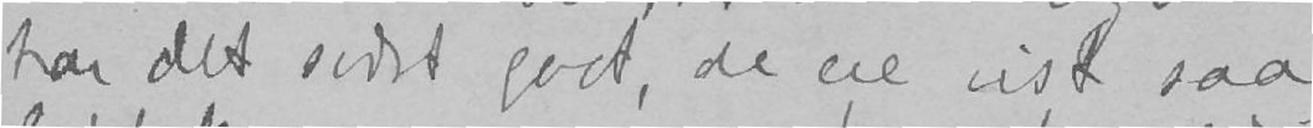har det svært godt, de ere vist saa
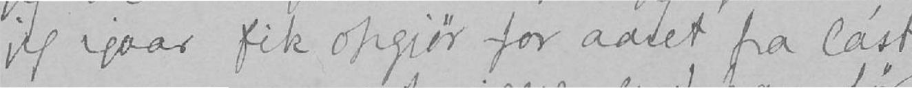jeg igaar fik opgjør for aaret fra Cast-
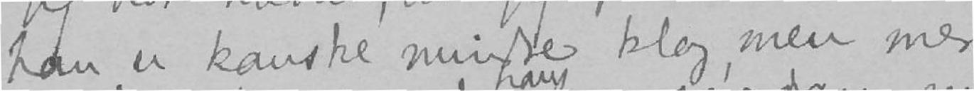han er kanske mindre klog, men mer
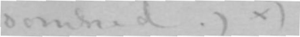somhed).*)
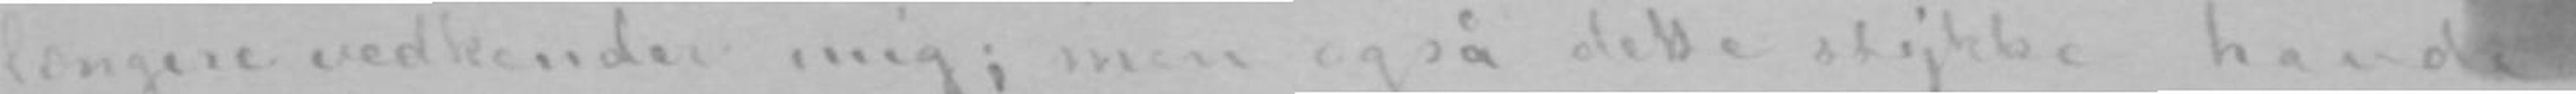længere vedkender mig; men også dette stykke havde
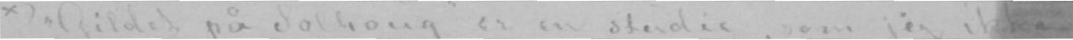*) «Gildet på Solhoug» er en studie, som jeg ikke
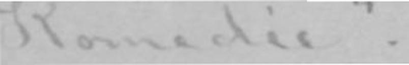Komedie».
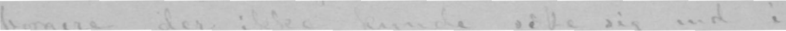borgere, der ikke kunde sætte sig ind i
